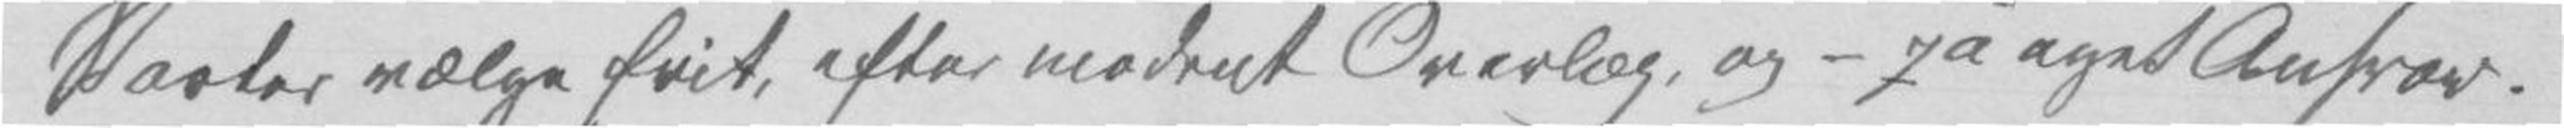Parter vælge frit, efter modent Overlæg, og – på eget Ansvar.
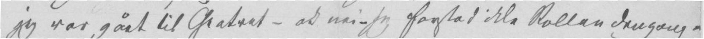jeg var gået til Theatret – ak nei – jeg forstod ikke Rollen dengang.
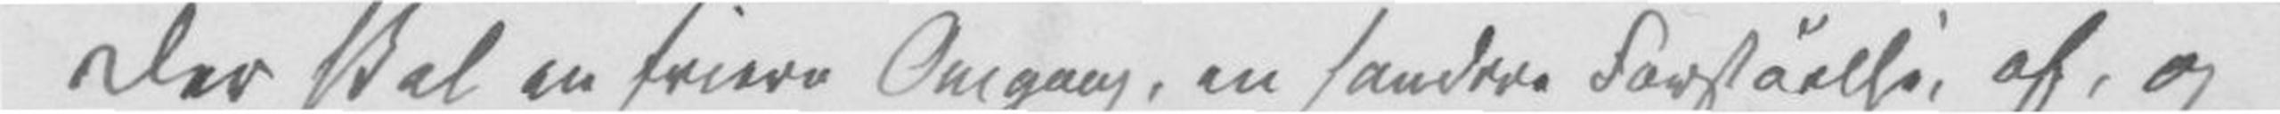Der skal en friere Omgang, en sandere Forståelse, af, og
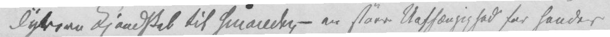dybere Kjendskab til hinanden – en større Uafhængighed for hendes
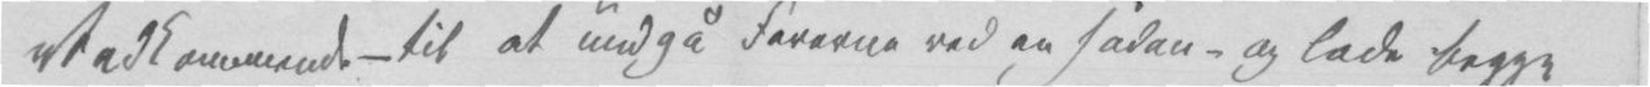Vedkommende – til at undgå Farerne ved en sådan – og lade begge
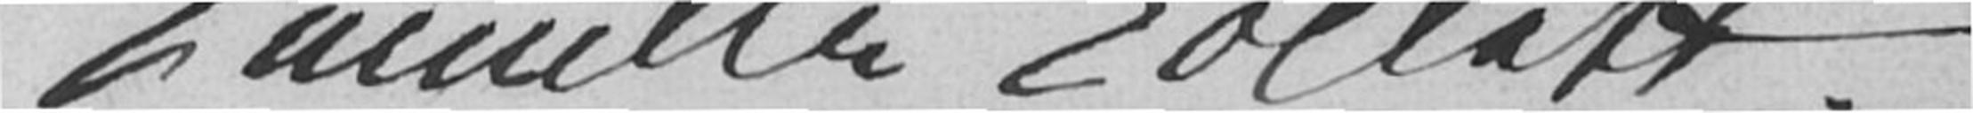Camilla Collett.
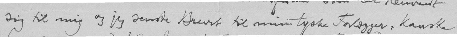sig til mig og jeg sendte Brevet til min tyske Forlægger, kanske
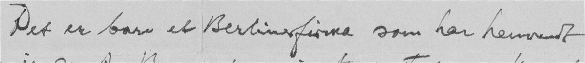Det er bare et Berlinsfirma som har henvendt
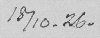18/10. 26.
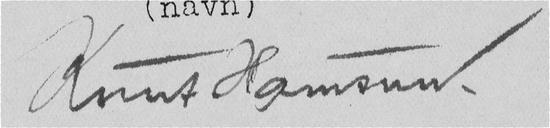Knut Hamsun.
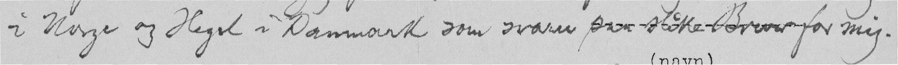i Norge og Hegel i Danmark som svarer paa slike Brever for mig.
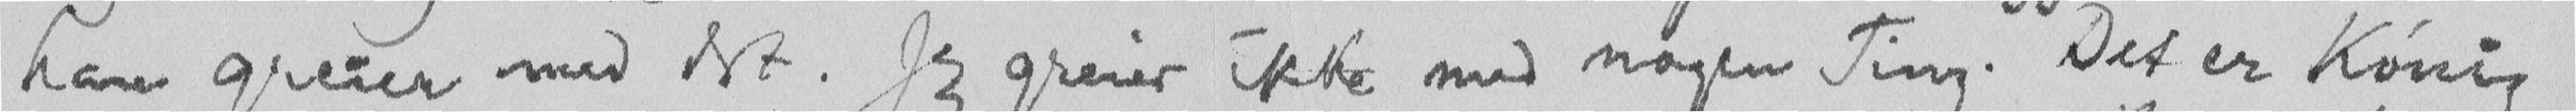han greier med det. Jeg greier ikke med nogen Ting. Det er Kønig
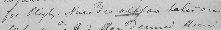for Pligt. Naar der altsaa tales om
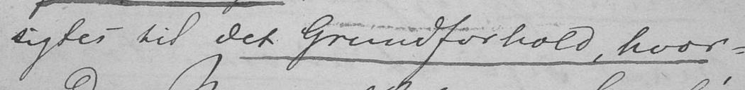sigtes til det Grundforhold, hvor-
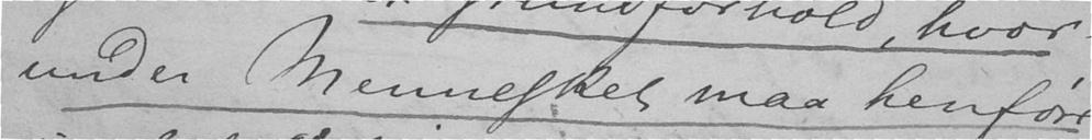under Mennesket maa henføre
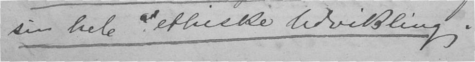sin hele ethiske Udvikling.
